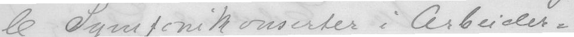le Symfonikonserter i Arbeider-
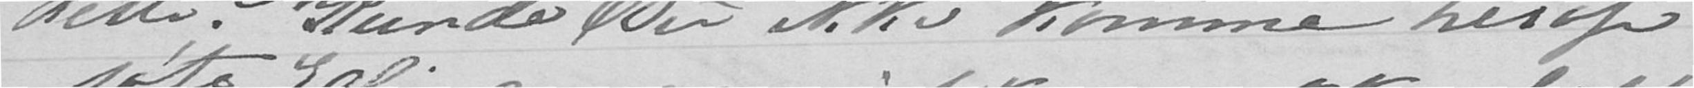dette? Kunde Du ikke kommer herop
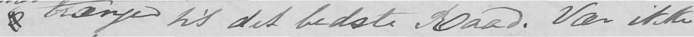og trænger til det bedste Raad. Vær ikke
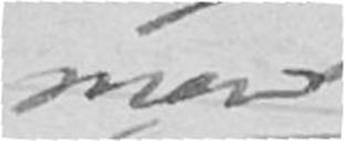men
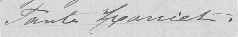Tante Harriet
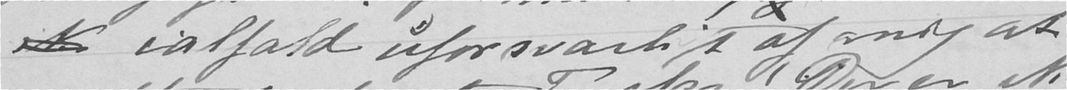ik ialfald uforsvarligt af mig at
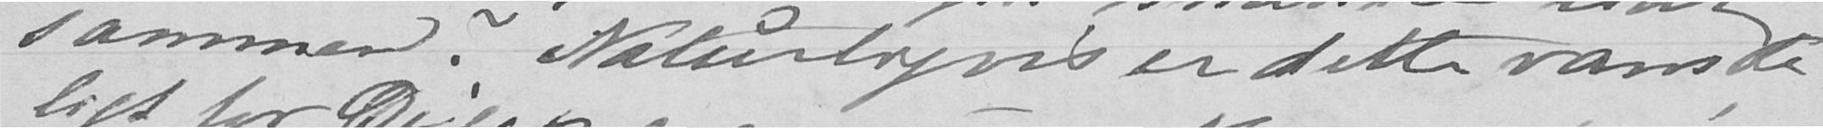sammen? Naturligvis er dette vanske-
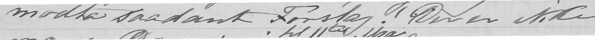modta saadant Forslag! Der er ikke
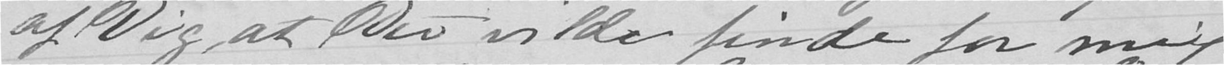af Dig, at Du vilde finde for mig
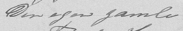Din egen gamle
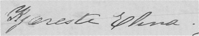Kjæreste Elina.
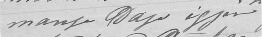mange Dage igjen
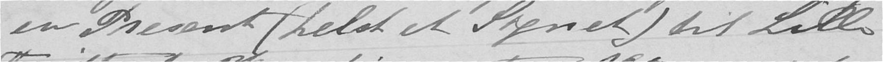en Present (helst et Signet) til Lille
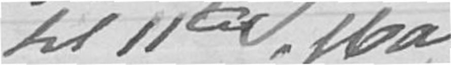til 11te
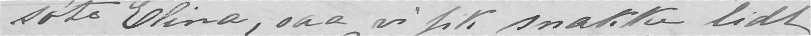søte Elina, saa vi fik snakke lidt
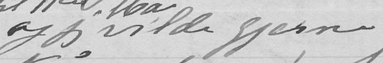og jeg vilde gjerne
